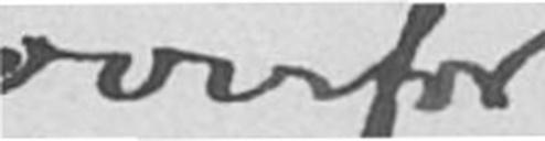overfor
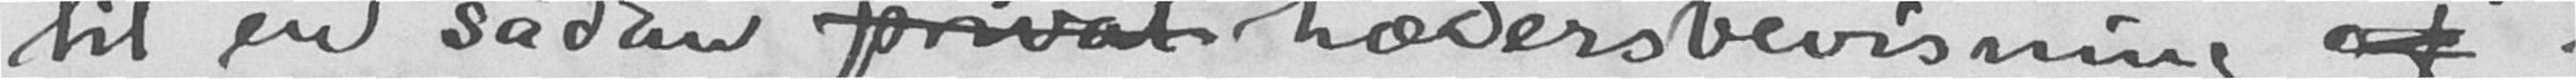til en sådan privat hædersbevisning af
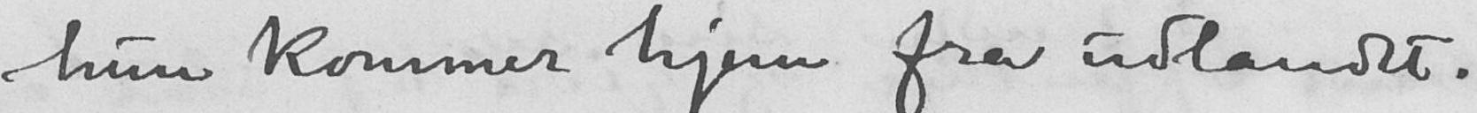hun kommer hjem fra udlandet.
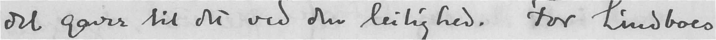del gaver  til det ved den leilighed. For Lindboes
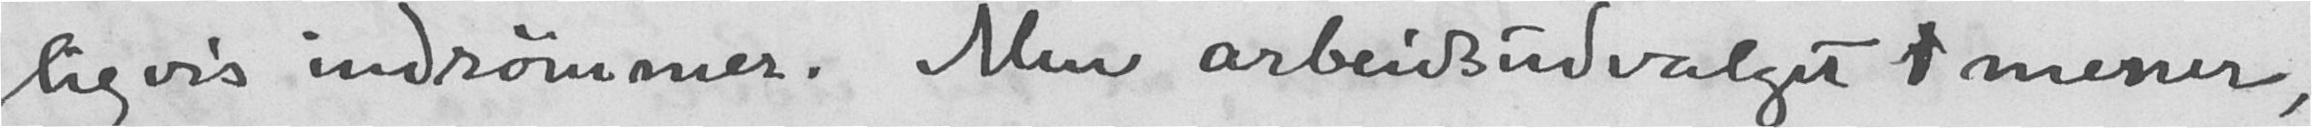ligvis indrømmer. Men arbeidsvalget i mener,
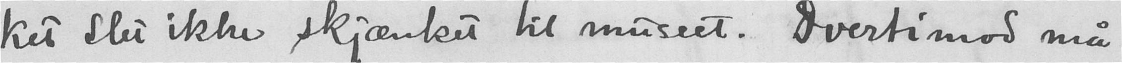ket slet ikke skjænket til museet. Tvertimod må
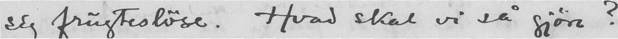seg frugtesløse. Hvad skal vi så gjøre?
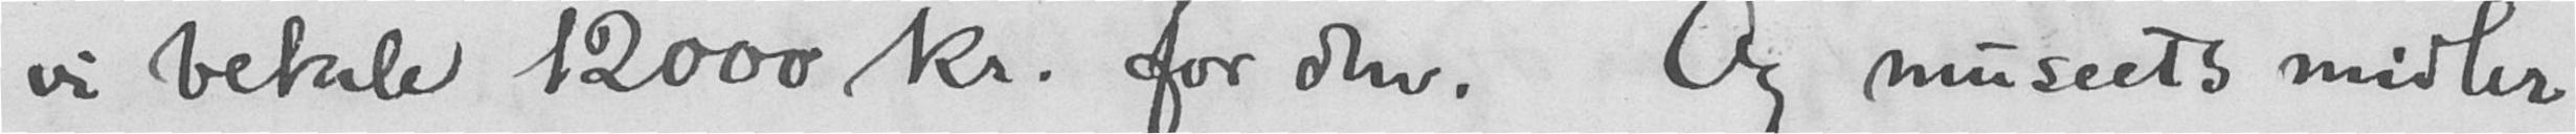vi betale 1200 kr. for den. Og museets midler
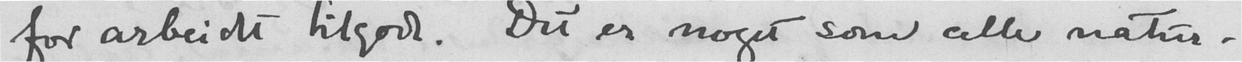for arbeidet tilgode. Det er noget som alle natur-
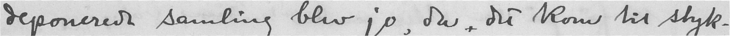deponerede samling blev jo, da, det kom til styk-
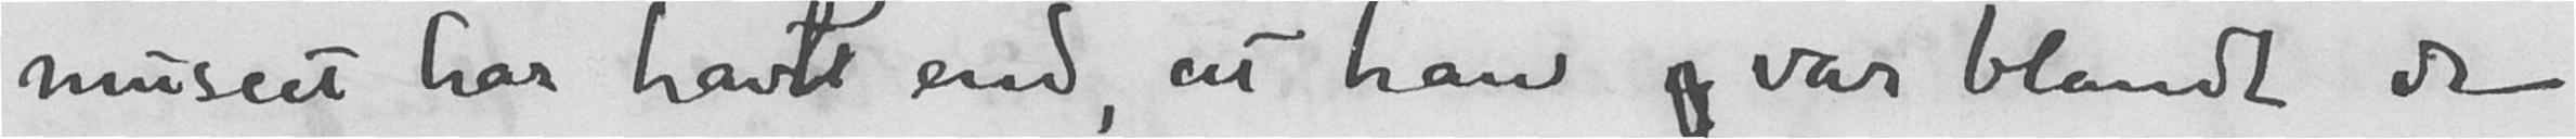museet har havt end, at han var blandt de
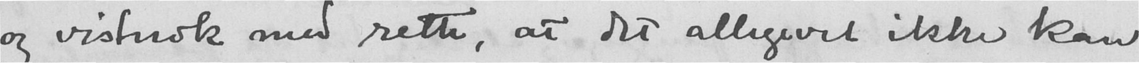og visktnok med rette, at det alligevel ikke kan
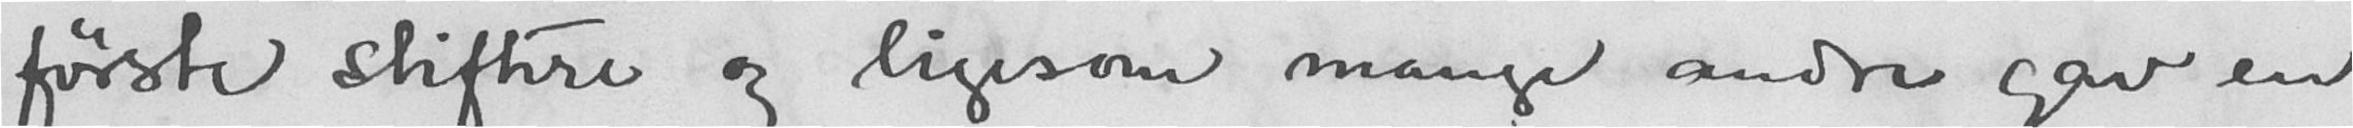første stiftere og ligesom mange andre gav en
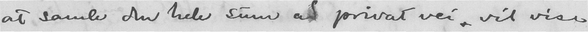at samle den hele sum ad privat vei, vil vise
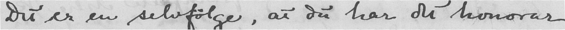Det er en selvfølge, at du har det  honorar
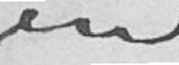en
